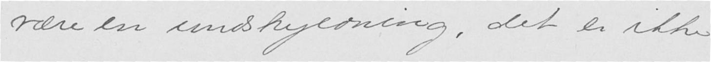være en undskyldning, det er ikke
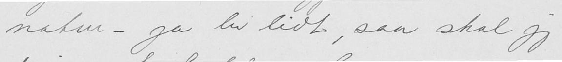natur - ja bi lidt, saa skal jeg
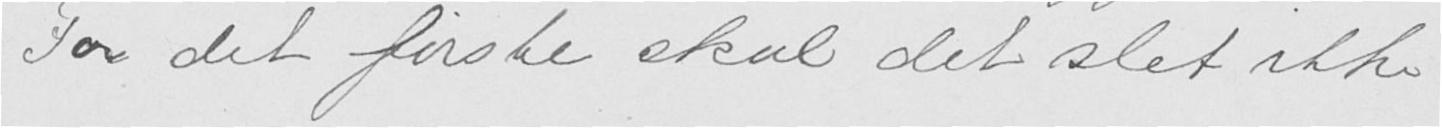For det første skal det slet ikke
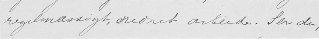regelmæssigt, ordnet arbeide. Ser du,
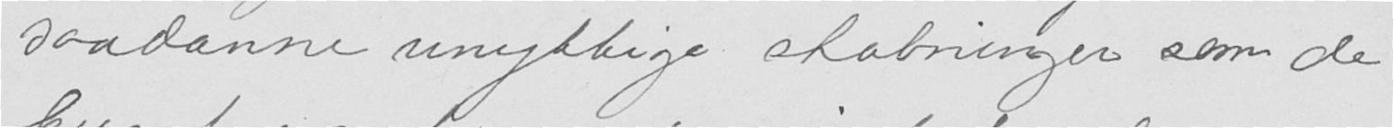saadanne unyttige skabninger som de
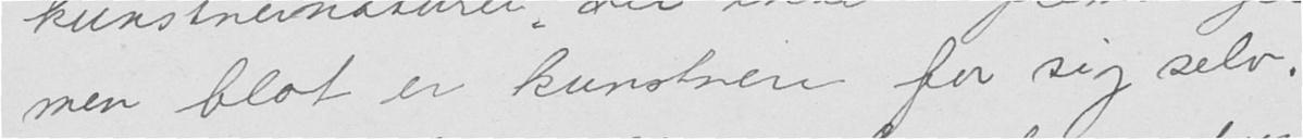men blot er kunstnere for sig selv.
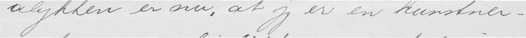lykken er nu, at jeg er en kunstner-
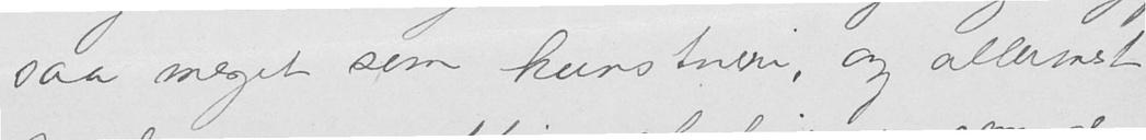saa meget som kunstnere, og allermest
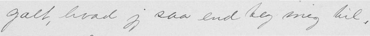galt, hvad jeg saa end tog mig til,
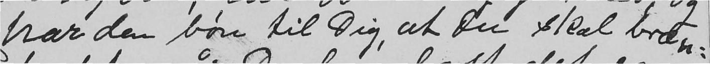har den bøn til Dig, at Du skal bræn-
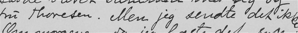fru Thoresen. Men jeg sendte det ikke.
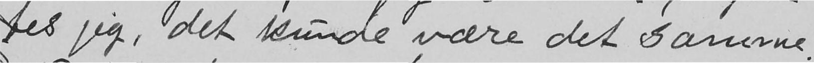tes jeg, det kunde være det samme.
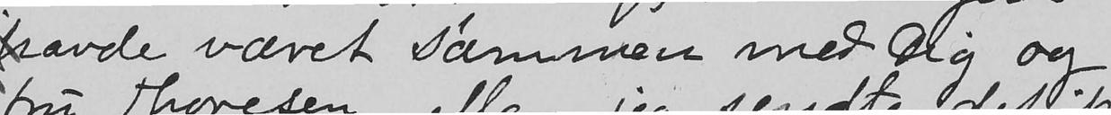havde været sammen med Dig og
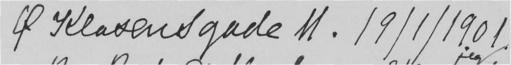Ø Klasendgade 11. 19/1/1901.
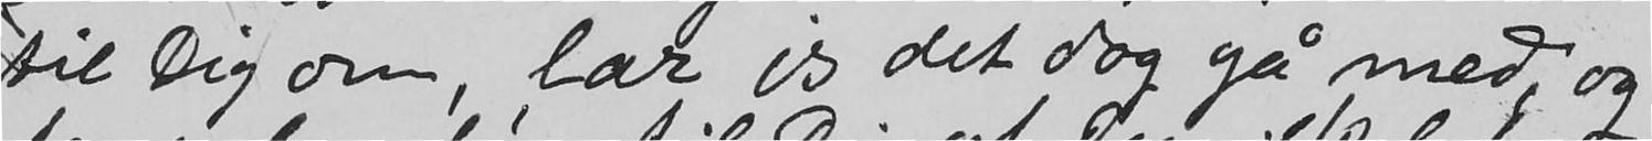til Dig om, lar jeg det dog gå med, og
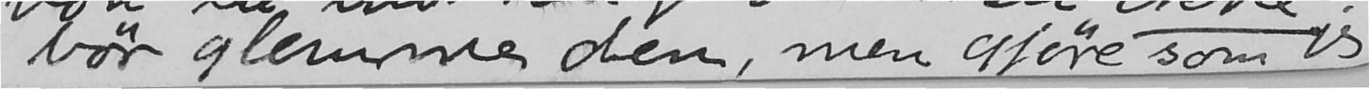bør glemme den, men gjøre som jeg
